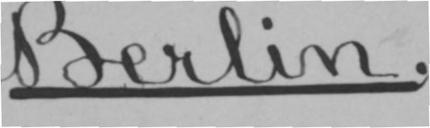Berlin.
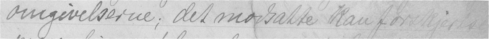omgivelserne; det modsatte kan forskjert
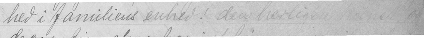hed i famliens enhed! den herligste kunst og
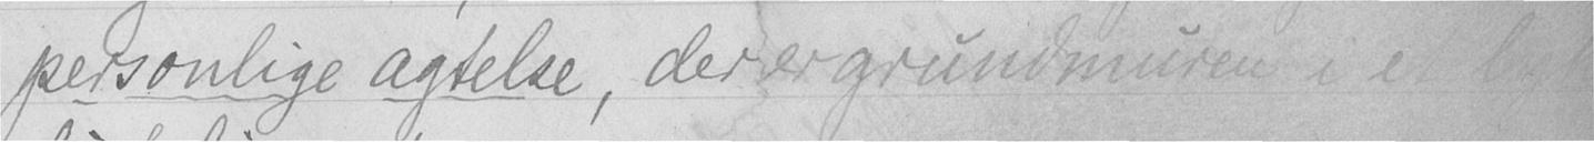personlige agtelse, der er grundmuren i et b
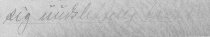sig uudslettelig fast i dem og
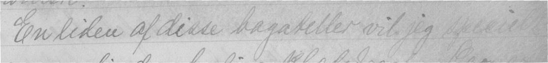En liden af disse bagateller vil jeg specielt
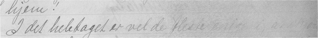I det heletaget er vel de fleste enige i, at skjøn-
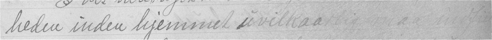heden inden hjemmet uvilkaarlig maa indfine
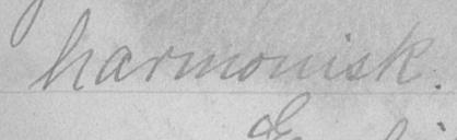harmonisk.
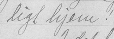ligt hjem!
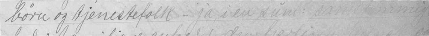børn og tjenestefolk - ja, i en sum: samstemmig-
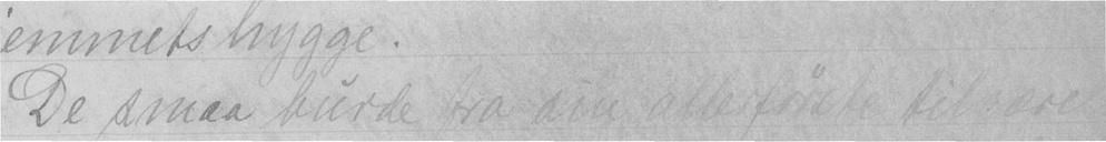De smaa burde fra sin allerførste tilvære
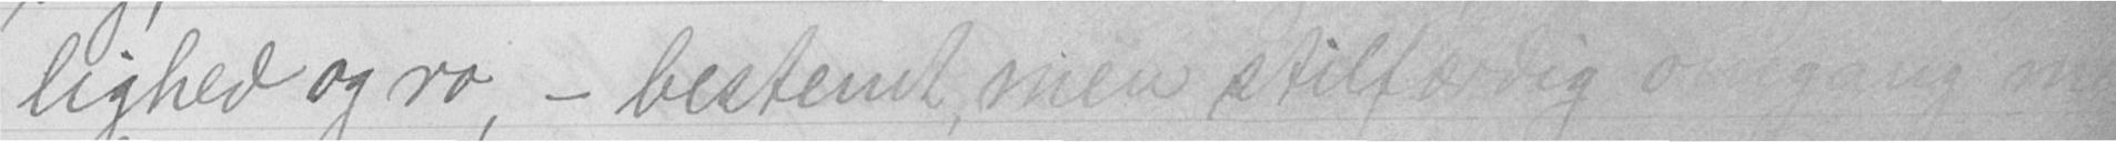lighed og ro, - bestemt, men stilfærdig omgang med
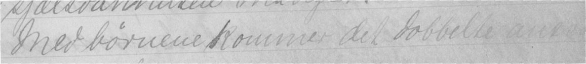Med børnene kommer det dobbelte ansvar
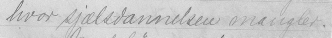hvor sjælsdannelsen mangler.
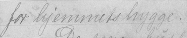for hjemmets hygge.
